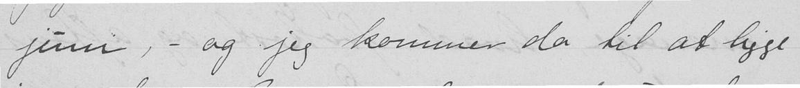juni, - og jeg kommer da til at ligge
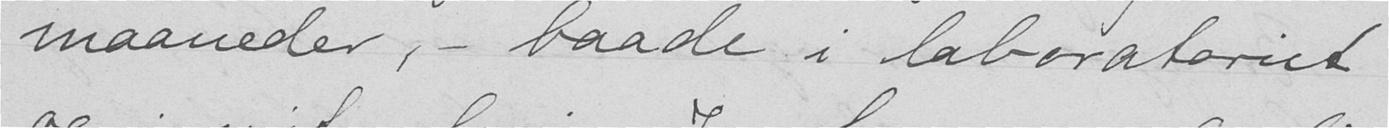maaneder, - baade i laboratoriet
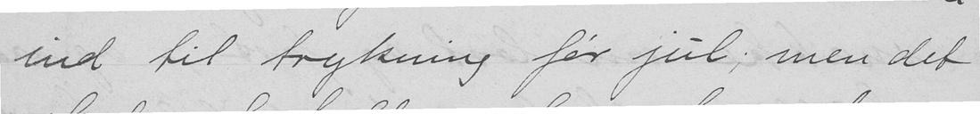ind til trykning før jul; men det
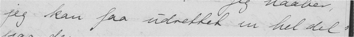jeg kan faa udrettet en hel del
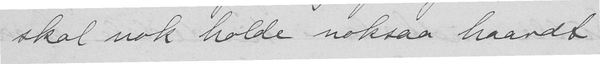skal nok holde noksaa haardt.
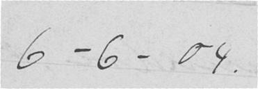6-6-04.
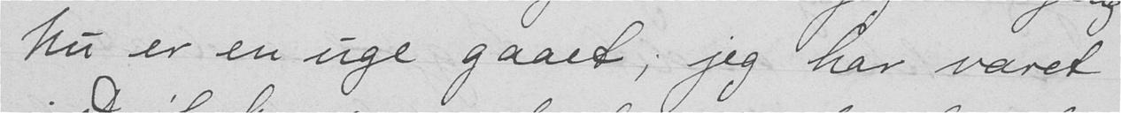Nu er en uge gaaet; jeg har været
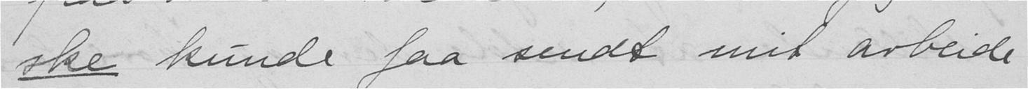ske kunde faa sendt mit arbeide
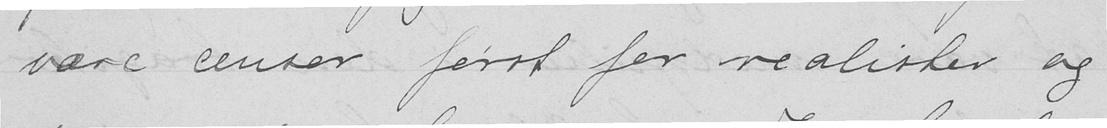være censor først for realister og
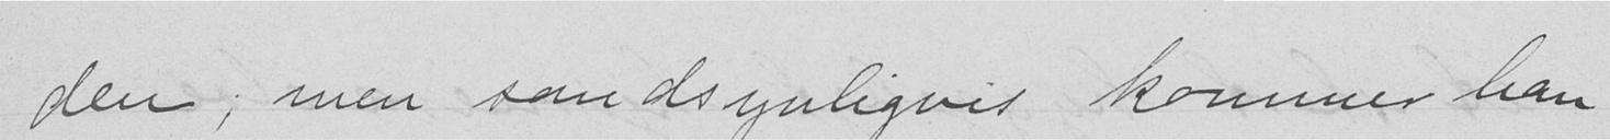den; men sandsynligvis kommer han
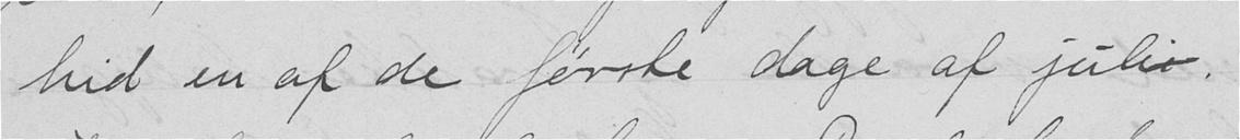hid en af de første dage af juli.
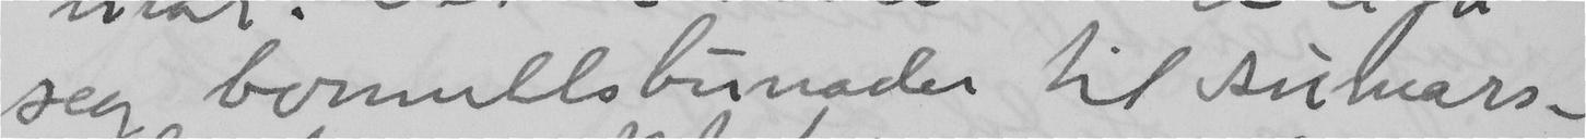seg bomullsbunader til sumars-
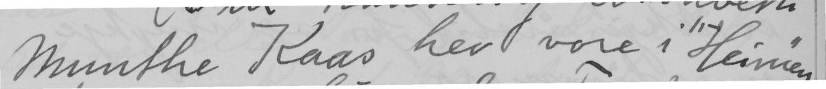Munthe Kaas hev vore i "Heimen"
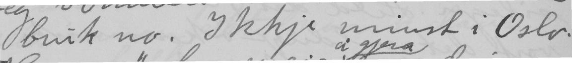bruk no. Ikkje minst i Oslo.
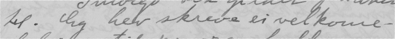til. Eig hev skreve ei velkome-
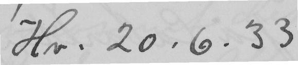Hv. 20.6.33
